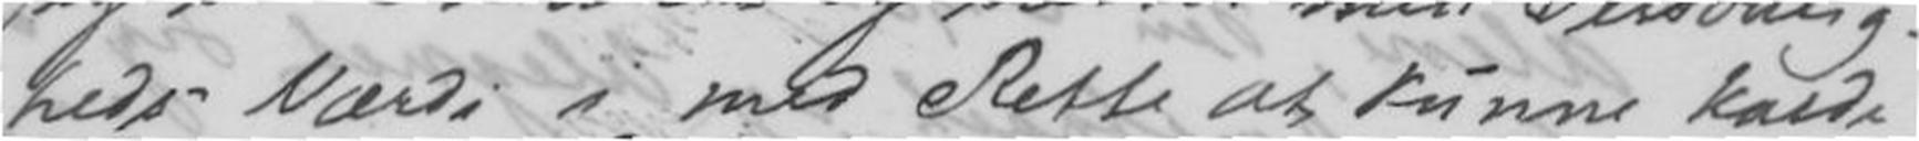heds Værdi i med Rette at kunne kalde
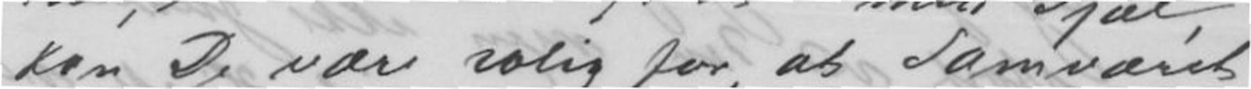kan De være rolig for, at Samværet
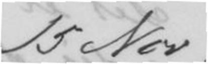15 Nov
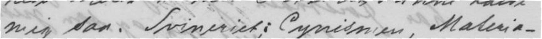mig saa. Svineriet, i Cynismen, Materia-
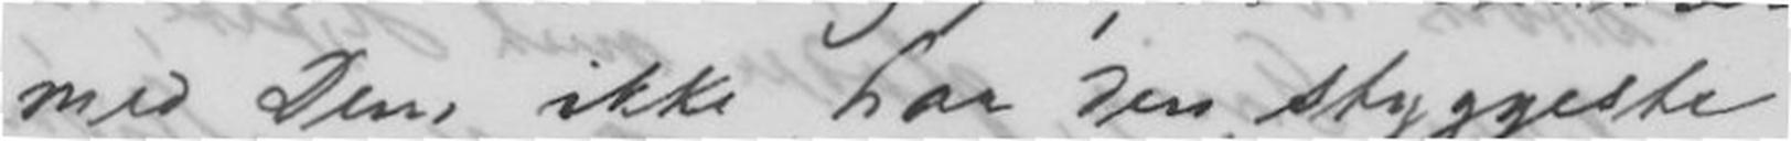med Dem ikke har den styggeste
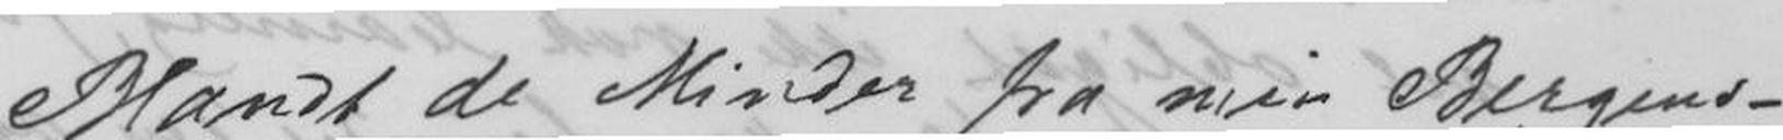Blandt de Minder fra min Bergens-
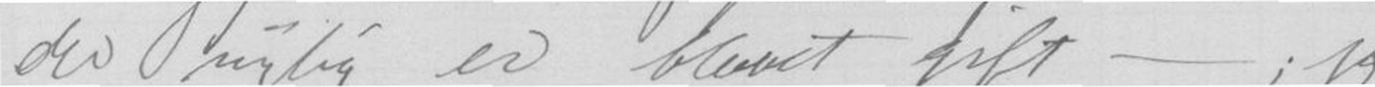der nylig er blevet gift -; jeg
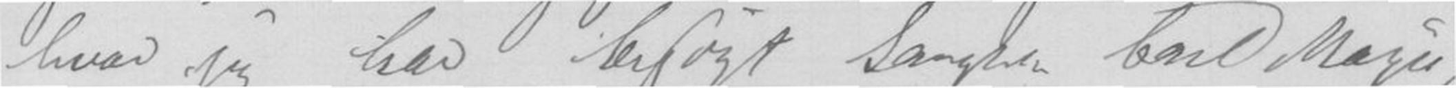hvad jeg har besøgt Sangeren Carl Mayer,
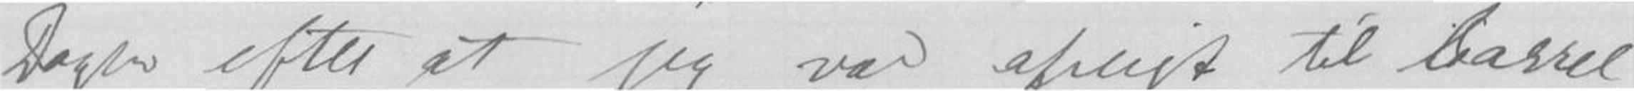Dagen efter at jeg var afreist til Cassel,
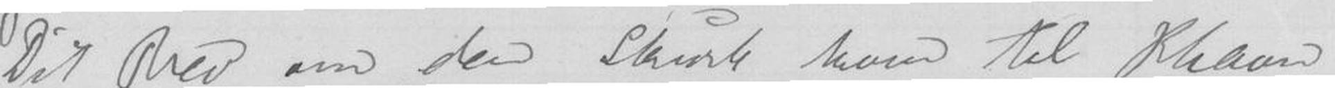Dit Brev om den skurk kom til Khavn
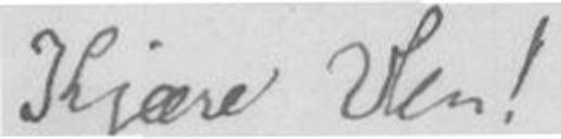Kjære Ven !
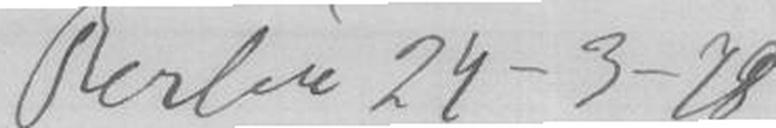Berlin 24-3-78
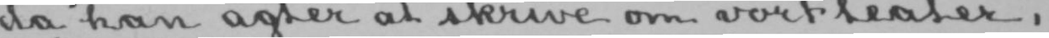da han agter at skrive om vort teater,
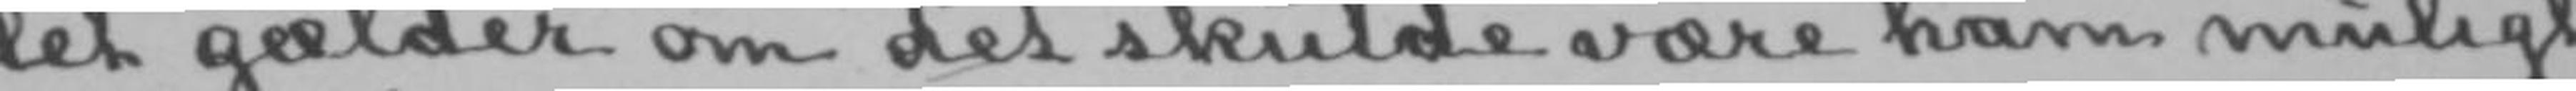let gælder om det skulde være ham muligt
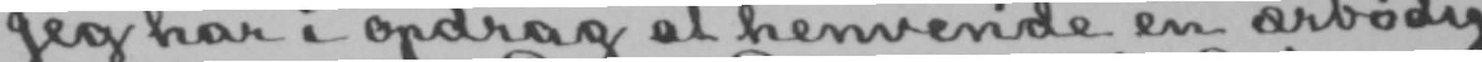Jeg har i opdrag at henvende en ærbødig
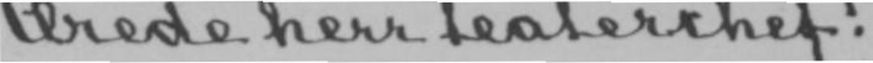Ærede herr teaterchef!
